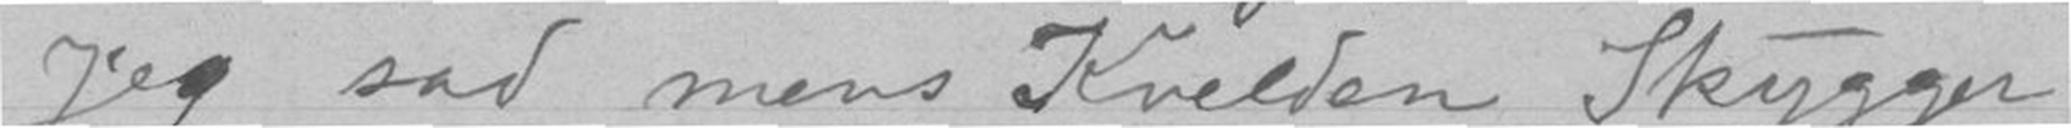Jeg sad mens Kvelden Skygger
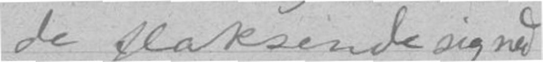de flaksende sig ned
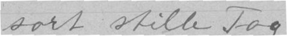sort stille Tog
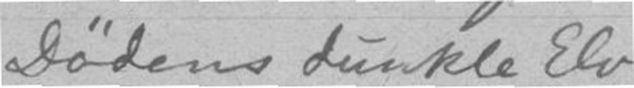Dødens dunkle Elv
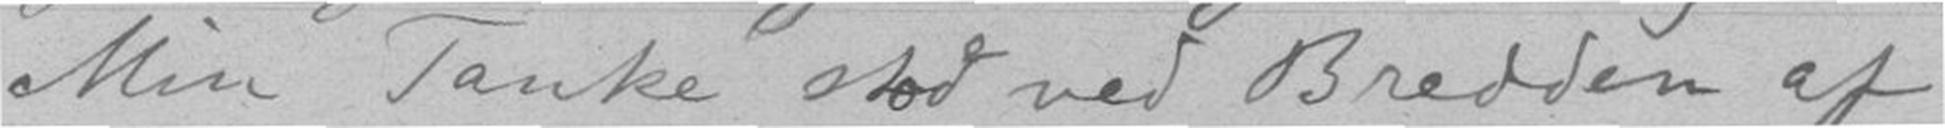Min Tanke stod ved Bredden af
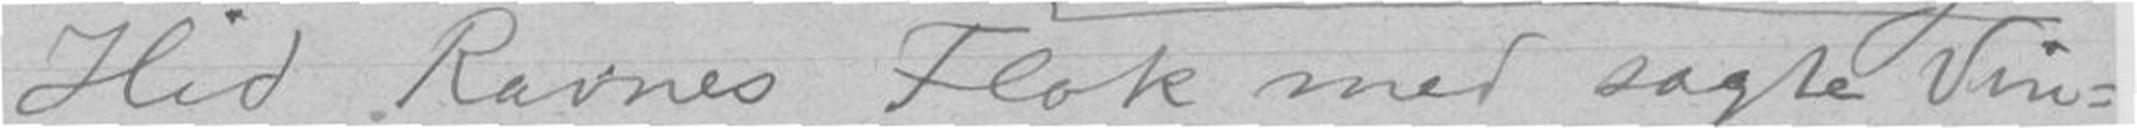Hid Ravnes Flok med sagte Vin-
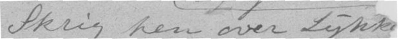Skrig han over Lykke
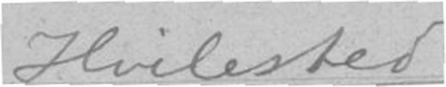Hvilested
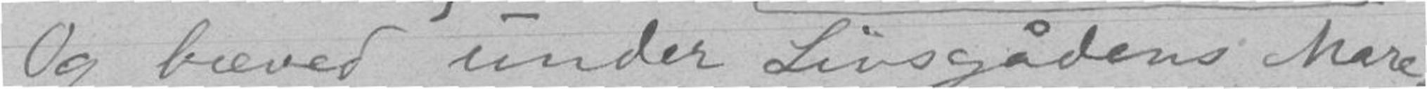Og bæved' under Livsgådens Mare.
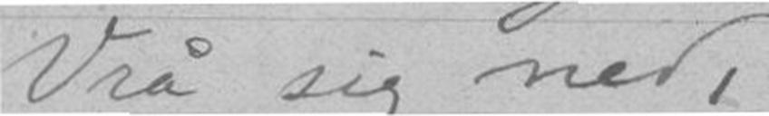Vrå sig ned.
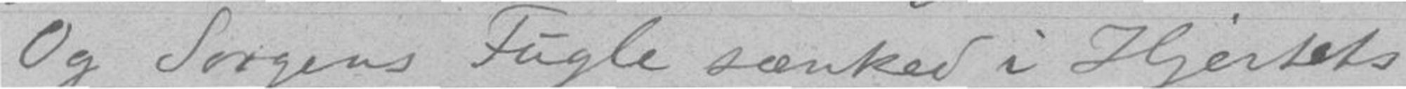Og Sorgens Fugle sænker i Hjertets
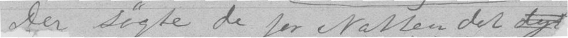Der søgte de for Natten det dy
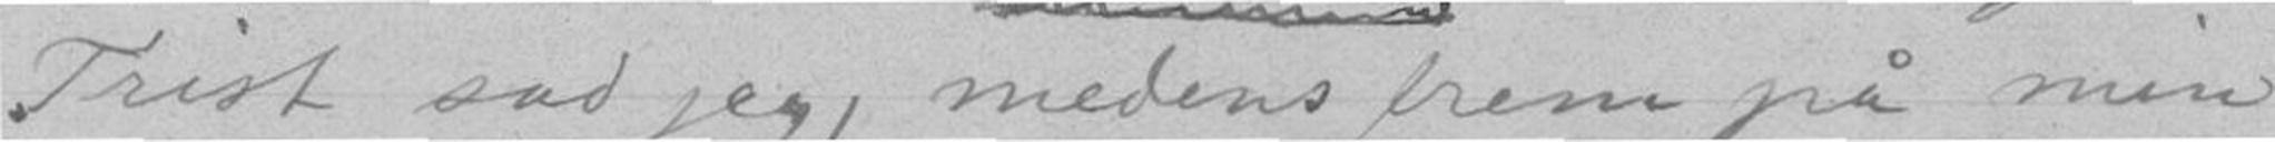Trist sad jeg, medens frem på min
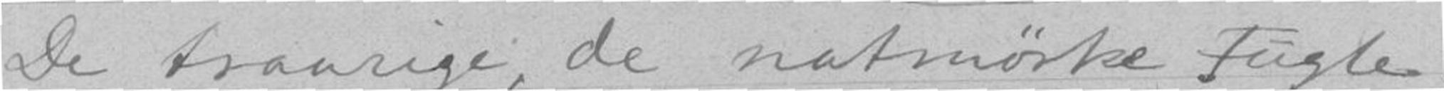De traurige, de natmørke Fugle,
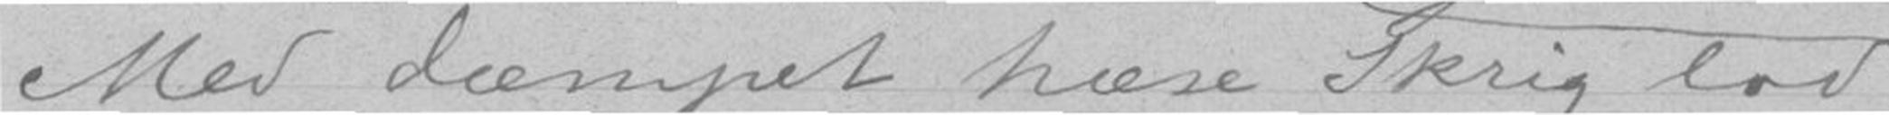Med dæmpet hæse Skrig lod
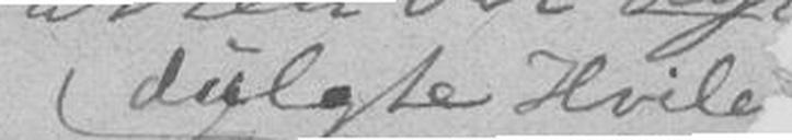dulgte Hvile
